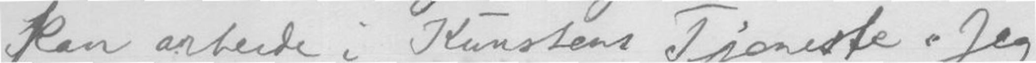kan arbeide i Kunstens Tjeneste. Jeg
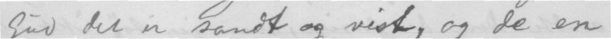Gud det er sandt og vist, og de en
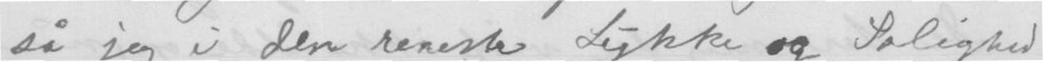så jeg i den reneste Lykke og Salighed
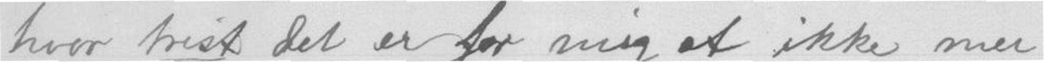hvor trist det er for mig at ikke mer
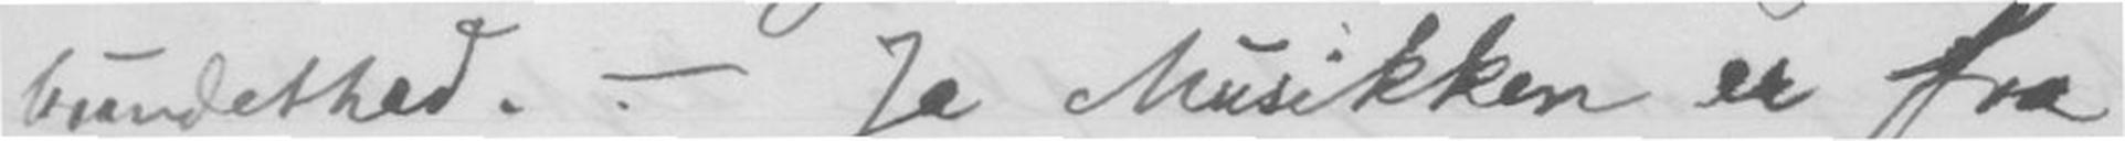bundethed. - Ja Musikken er fra
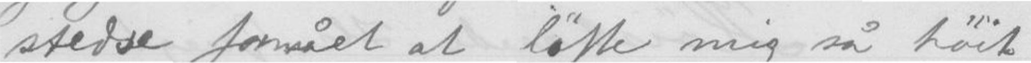stedse formået at løfte mig så høit
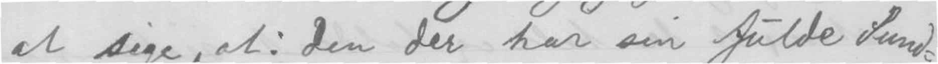at sige, at den der har sin fulde Sund-
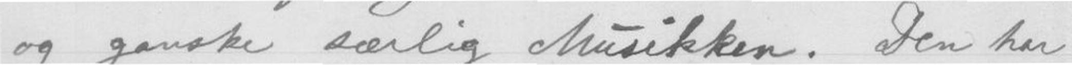og ganske særlig Musikken. Den har
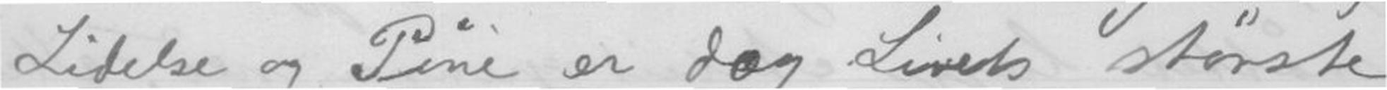Lidelse og Pine er dog Livets Største
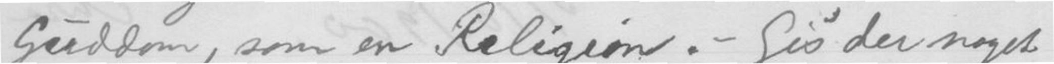Guddom, som en Religion. - Gis der noget
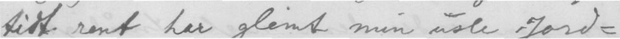tidt rent har glemt min usle Jord-
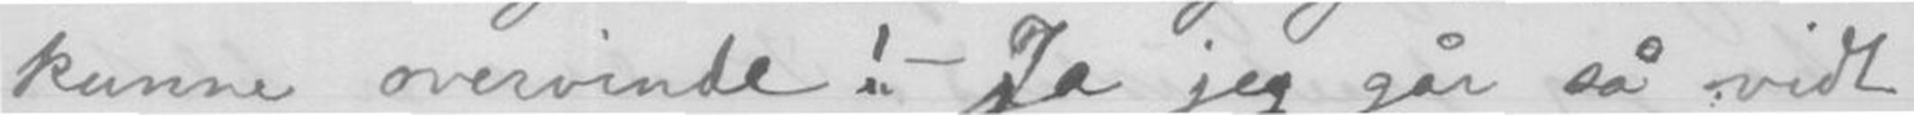kunne overvinde! - Ja jeg går så vidt
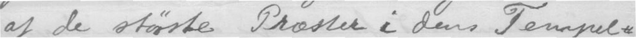af de største Præster i dens Tempel -
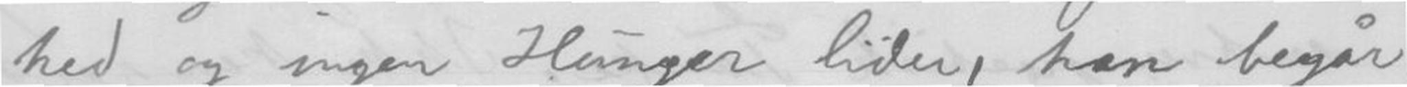hed og ingen Hunger lider, han begår
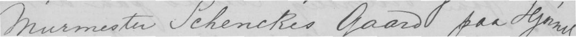Murmeister Schenckes Gaard paa Hjørnet
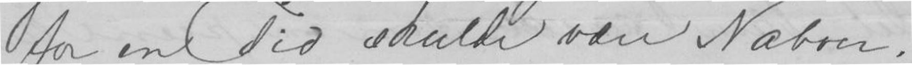for en Tid skulde være Naboer.
